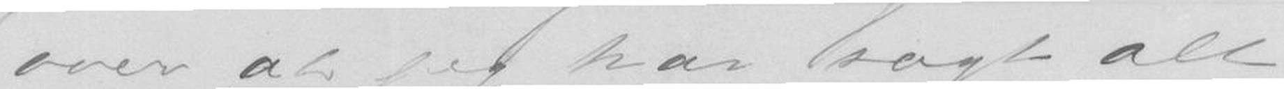over at jeg har sagt alt
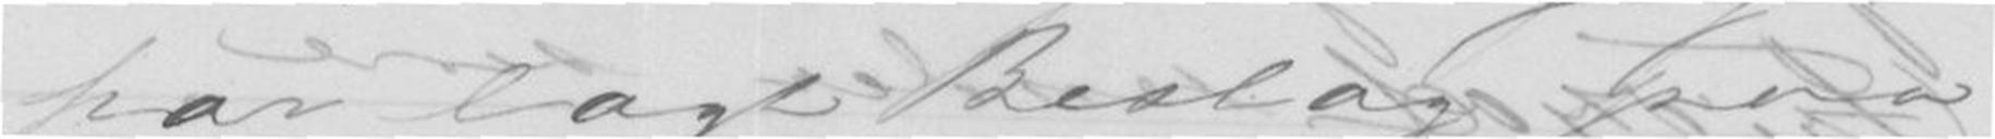har lagt Beslag paa
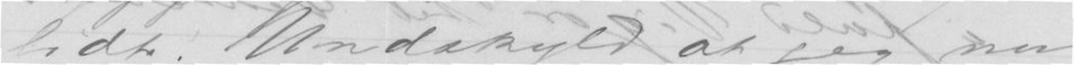lidt. Undskyld at jeg nu
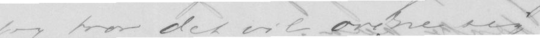jeg tror det vil ordne sig
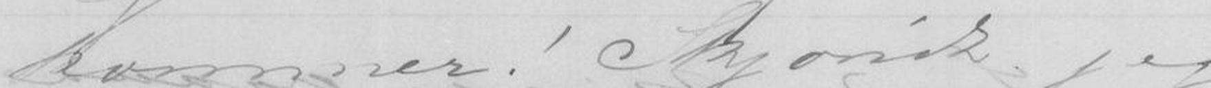kommer! Skjøndt jeg
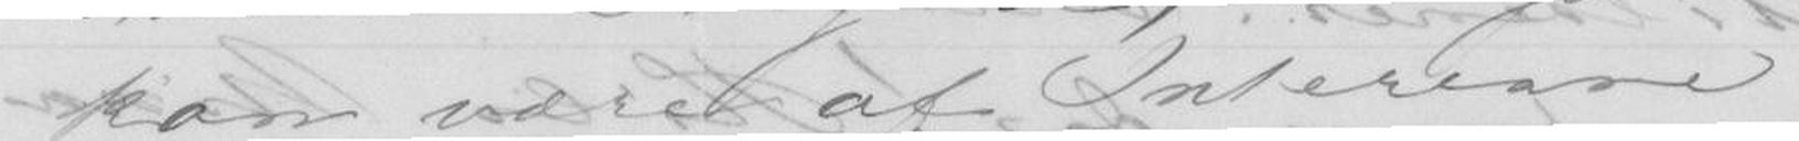kan være af Interesse
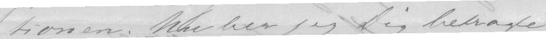tionen. Nu ber jeg Dig betragte
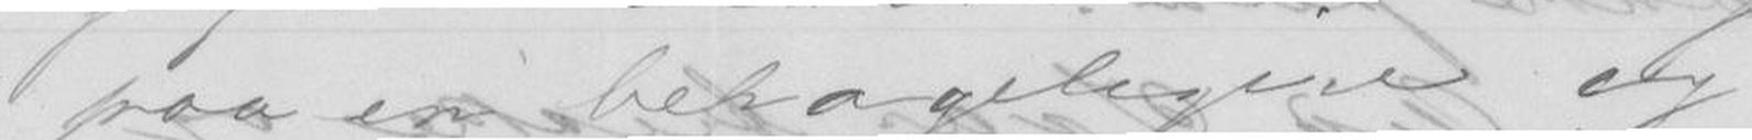paa en behageligere og
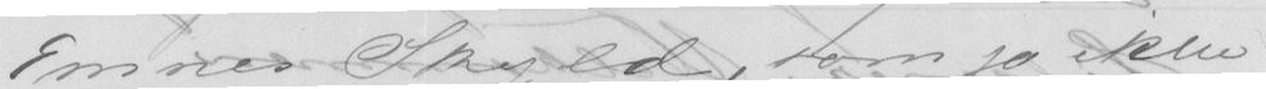Emnes Skyld, som jo ikke
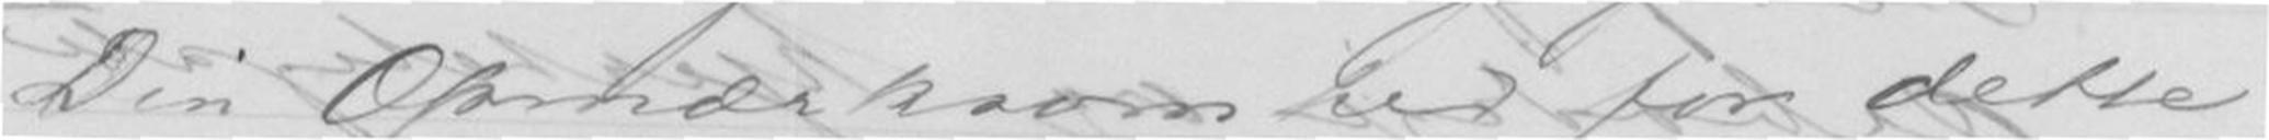Din Opmærksomhed for dette
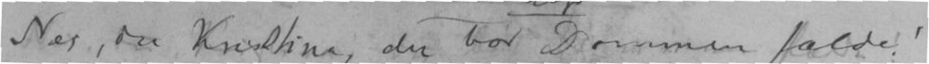Nei, du Kristina, der bør Dommen falde
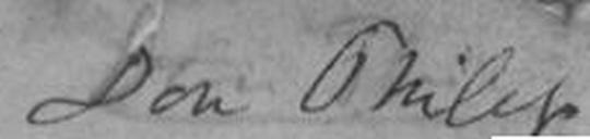Don Philip
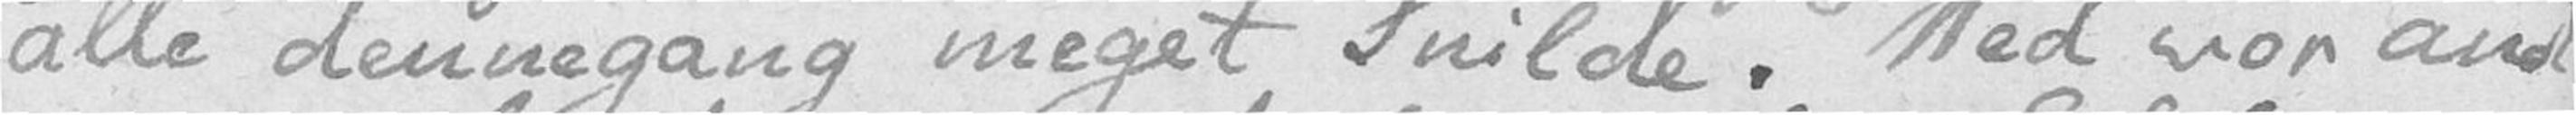alle dennegang meget Snilde. Ved vor and
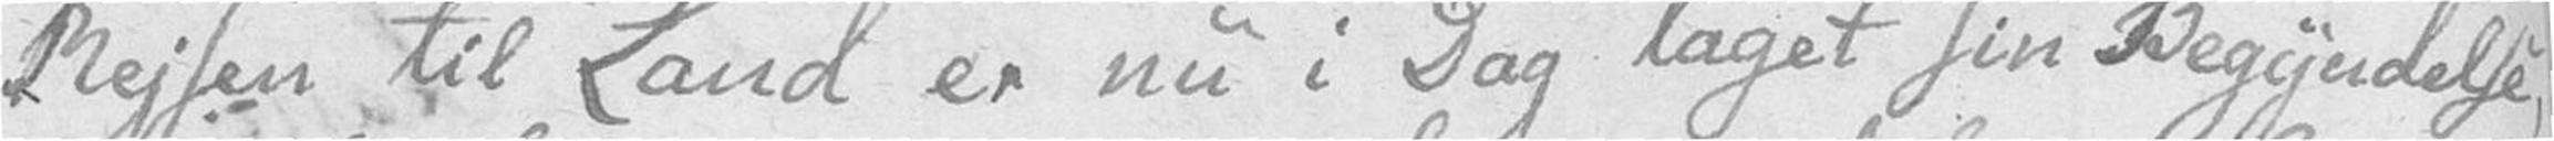Rejsen til Land er nu i Dag taget sin Begyndelse
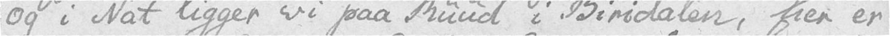og i Nat ligger vi paa Ruud i Biridalen, her er
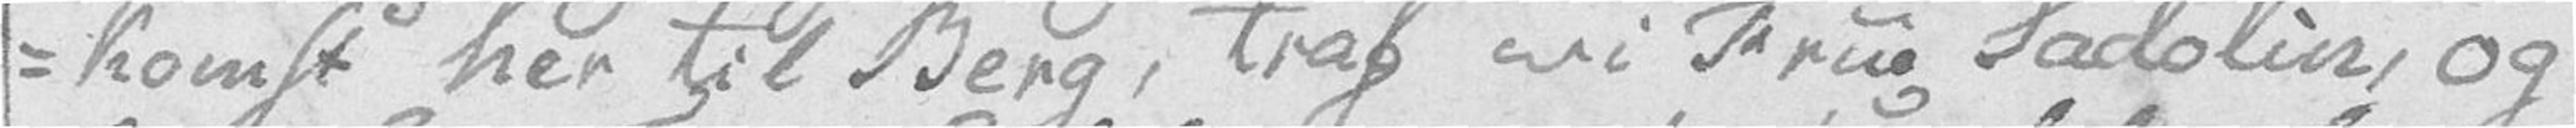-komst her til Berg, traf vi Frue Sadolin, og
